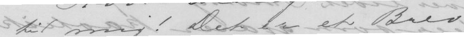til mig! Det er et Brev
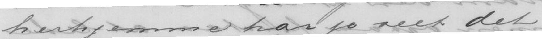herhjemme har jo seet det
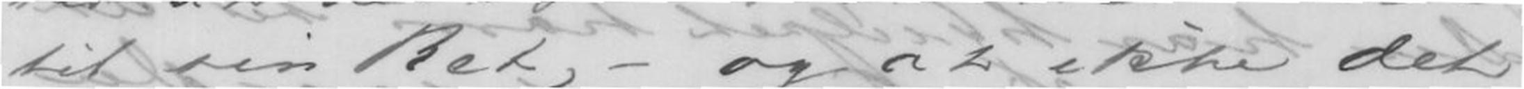til sin Ret,- og at ikke det
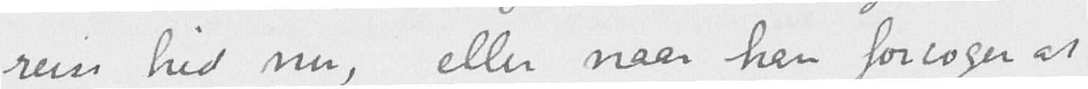reise hid nu, eller naar han forsøger at
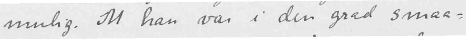mulig. At han var i den grad smaa-
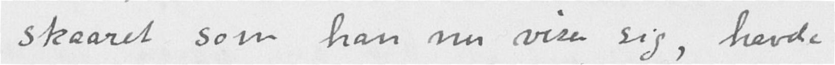skaaret som han nu viser sig, havde
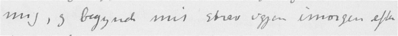mig, og begynde mit stræv igjen imorgen efter
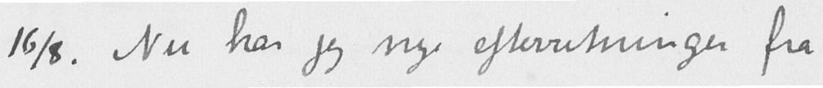16/8. Nu har jeg nye efterretninger fra
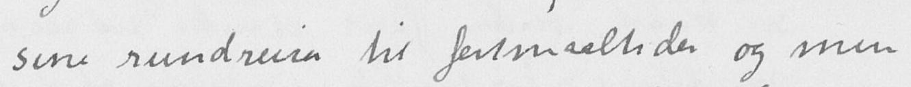sine rundreiser til festmaaltider og min
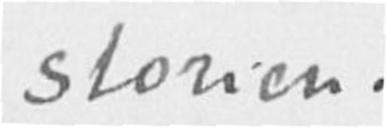storien.
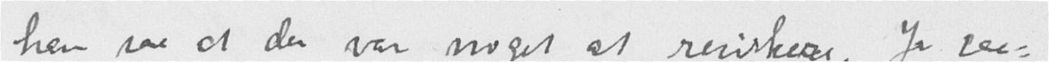han saa at der var noget at resirkerer. Ja saa-
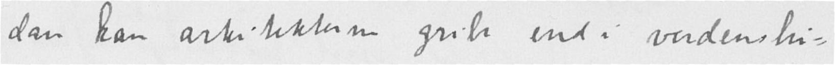dan kan arkitekterne gribe ind i verdenshi-
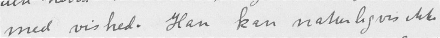med vished. Han kan naturligvis ikke
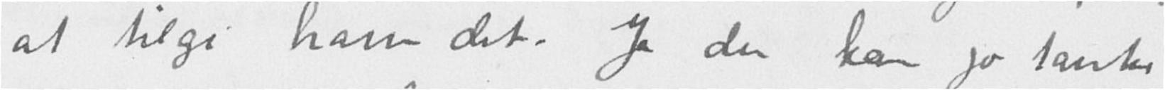at tilgi ham det. Ja du kan jo tænke
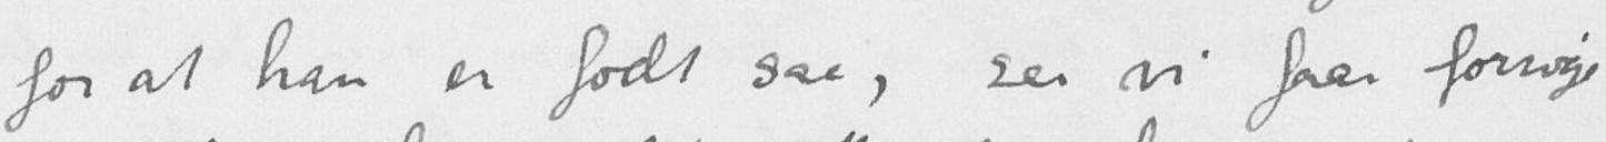for at han er født saa, saa vi faar forsøge
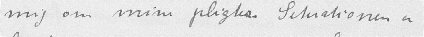mig om mine pligter. Situationen er
